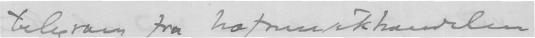telegram fra hofmusikhandelen
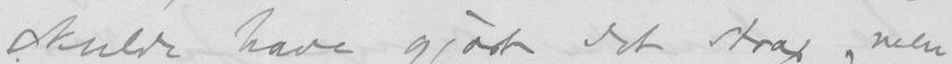skulde have gjort det strax, men
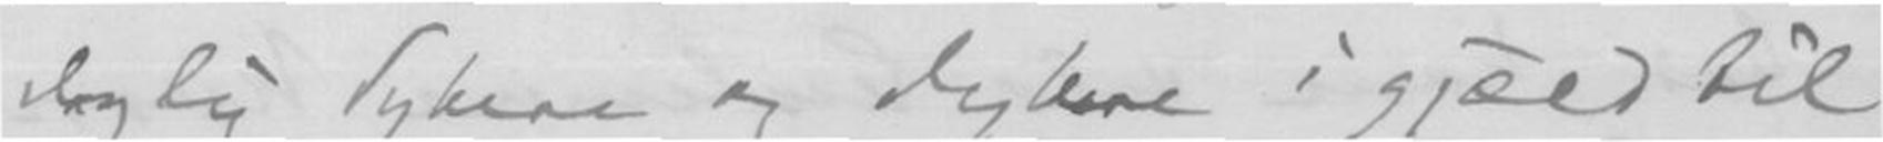daglig dybere og dybere i gjæld til
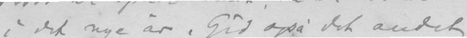i det nye år. Gid også det andet
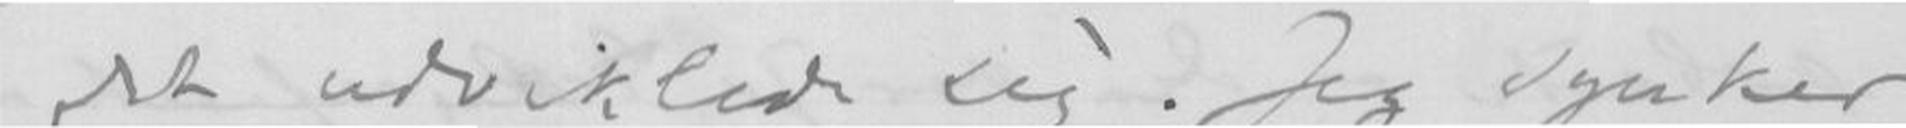det udviklede sig. Jeg synker
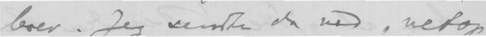brev. Jeg sendte da ned netop
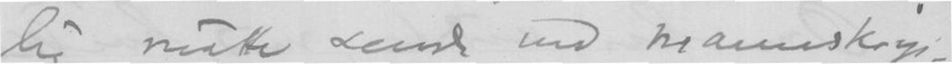lig måtte sende ned manuskrip-
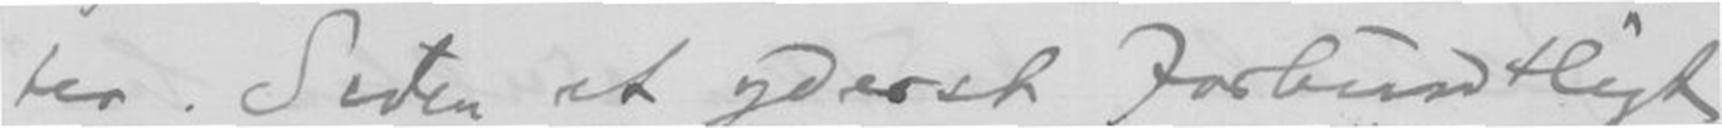ter. Siden et yderst forbundtligt
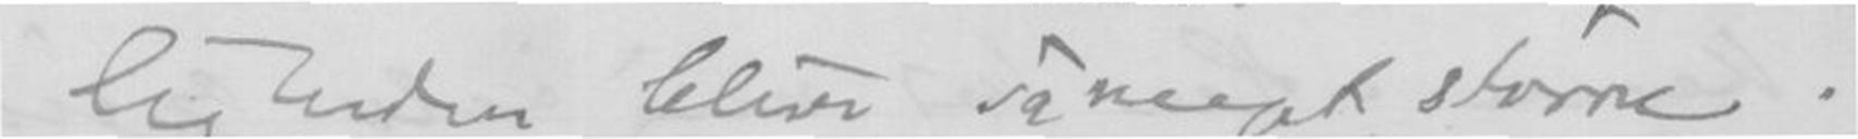ligheden blive så meget større.
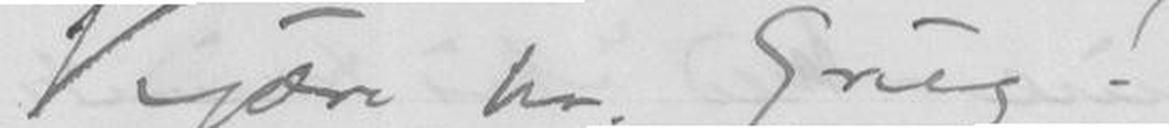Kjære hr. Grieg!
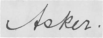Asker.
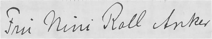Fru Nini Roll Anker
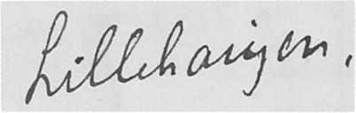Lillehaugen
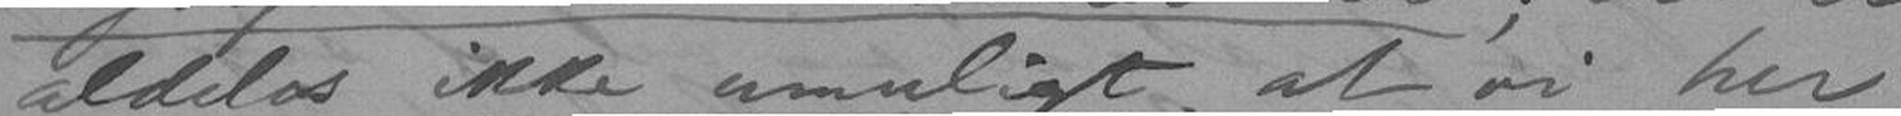aldeles ikke umuligt, at vi her
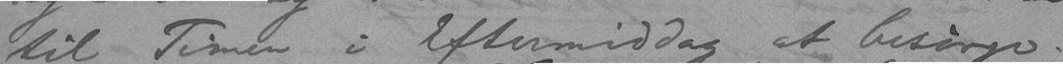til Timen i Eftermiddag at besørge.
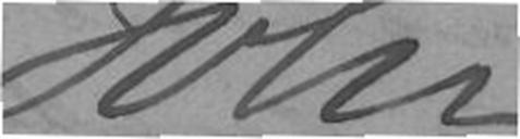John
